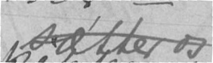sæ'tter os
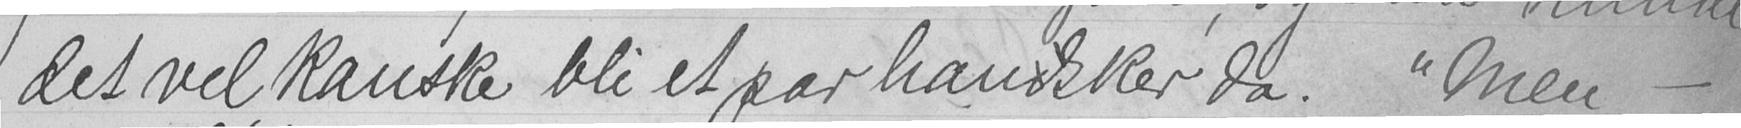det vel kanske bli et par handsker da. "Men -
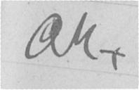Ak, Se
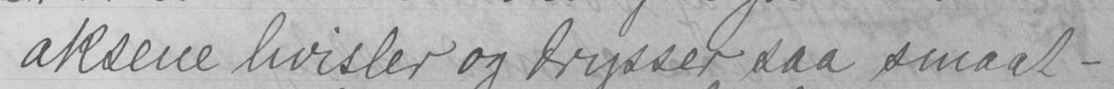aksene hvisler og drysser saa smaat -
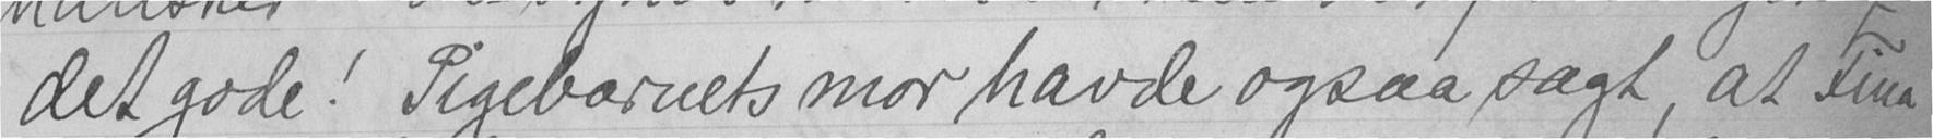det gode! Pigebarnets mor havde ogsaa sagt, at Tina
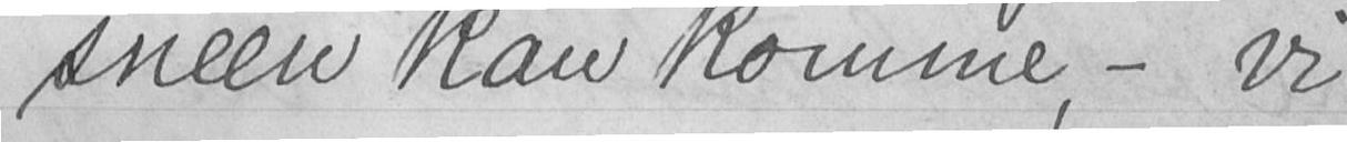sneen kan komme, - vi
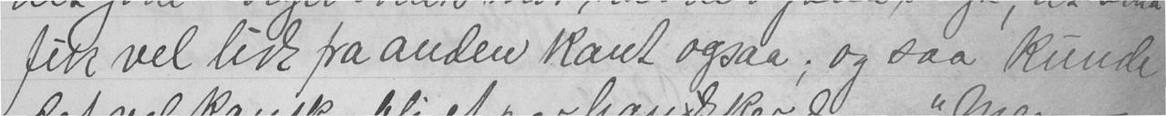fik vel lidt fra anden kant ogsaa; og saa kunde
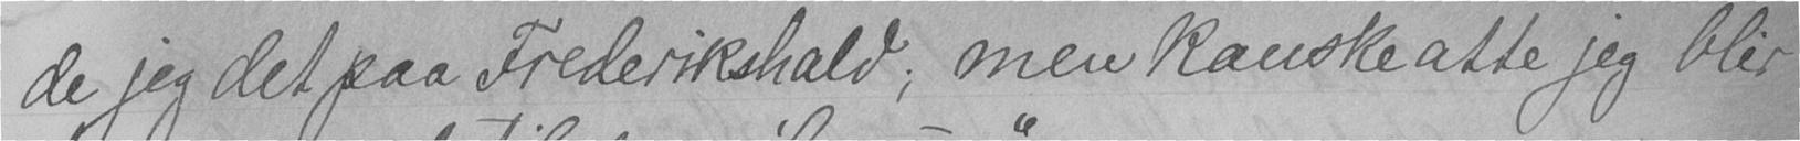de jeg det paa Frederikshald; men kanske atte jeg blir
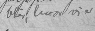blir, hvor vi er
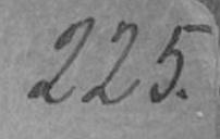225.
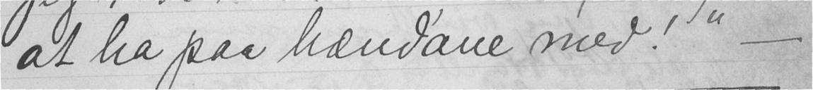at ha paa hænd'ane med! " -
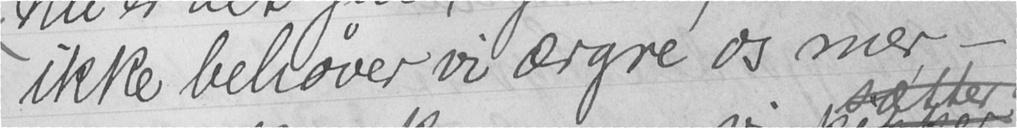ikke behøver vi ærgre os mer -
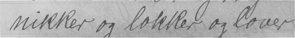nikker og lokker og lover
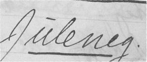Juleneg.
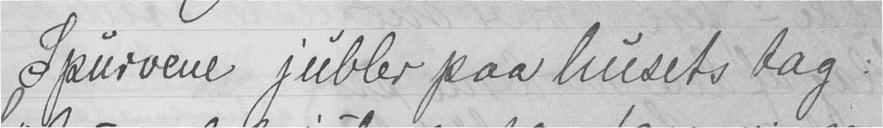Spurvene jubler paa husets tag:
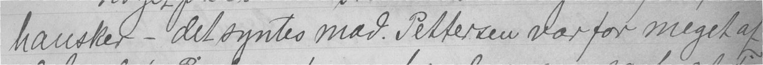hansker - det syntes mad. Pettersen var for meget af
